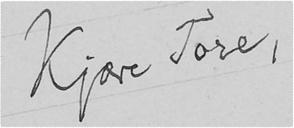Kjære Tore,
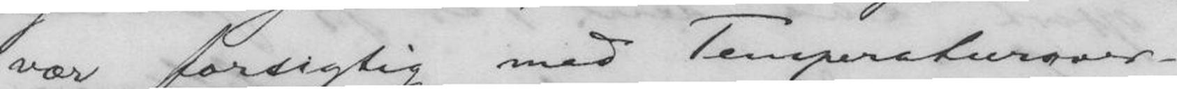vær forsigtig med Temperaturover-
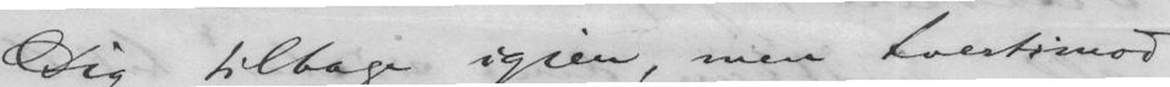Dig tilbage igjen, men tvertimod
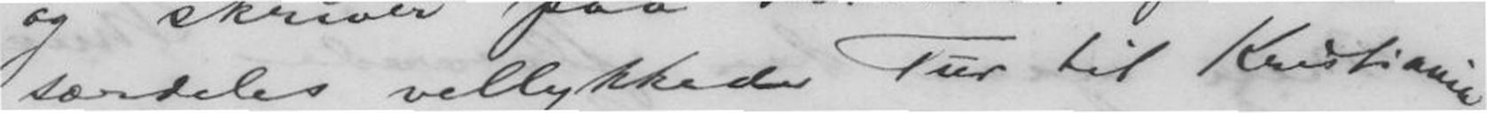særdeles vellykkede Tur til Kristiania.
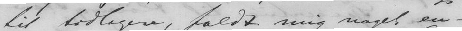til Tidlegere, faldt mig noget en-
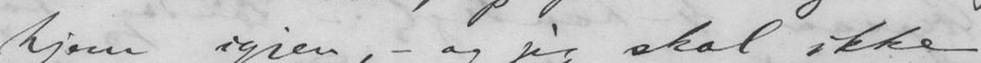hjem igjen, - og jeg skal ikke
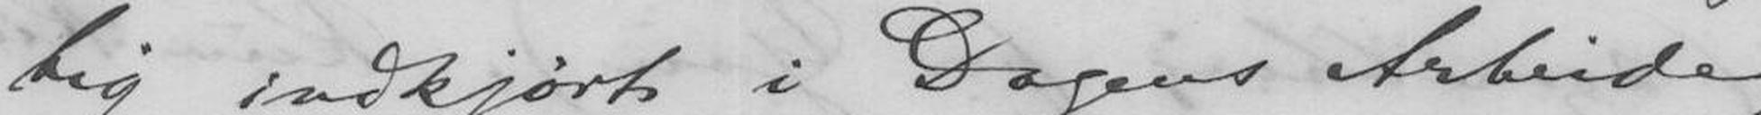tig indkjørt i Dagens Arbeide,
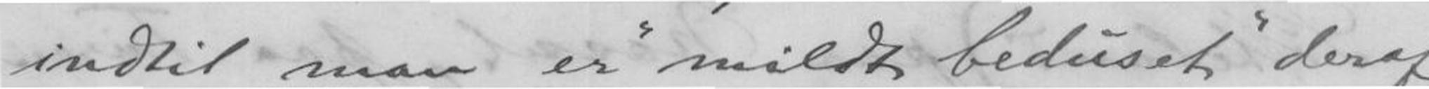indtil man er "mildt beduset" deraf.
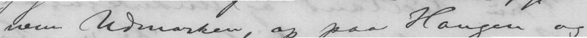nem Udmarken, op paa Haugen og
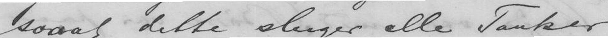saaat dette sluger alle Tanker
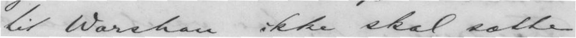til Warshau ikke skal sætte
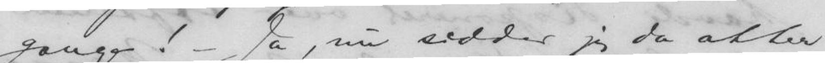gange! - Ja, nu sidder jeg da atter
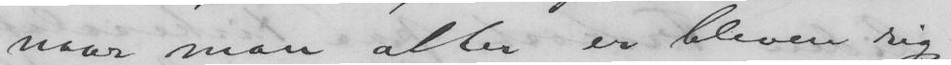naar man atter er bleven rig-
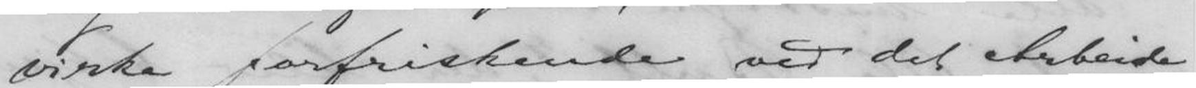virke forfriskende ved det Arbeide
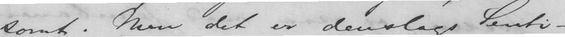somt. Men det er denslags Senti-
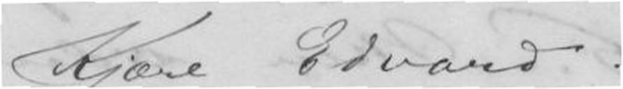Kjære Edvard!
